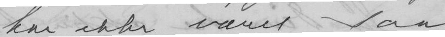har ikke været saa
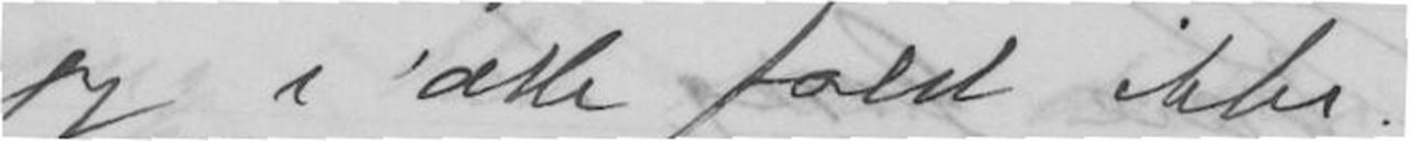jeg i alle fald ikke.
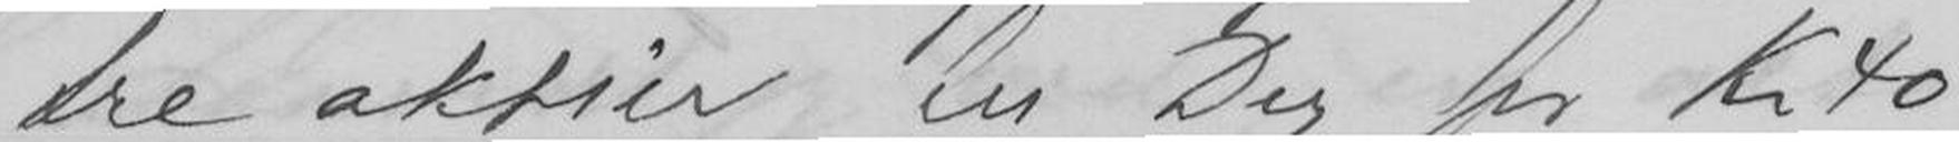tre aktier til Dig for kr 40
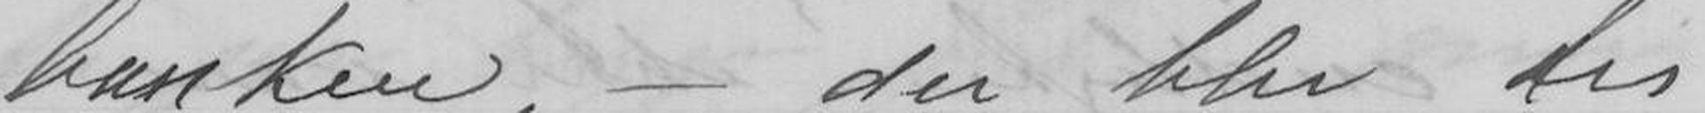banken, - der blev til
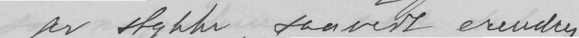pr stykke saavidt erindres
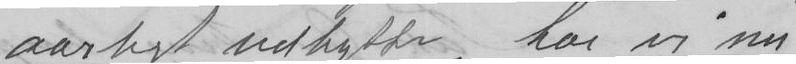aarligt udbytte, har vi nu
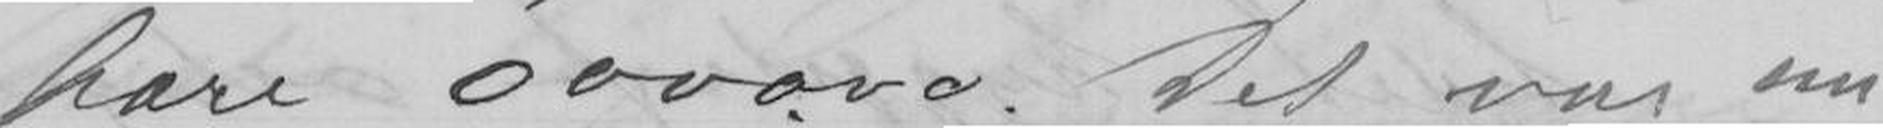bare 0000.00. Det var nu
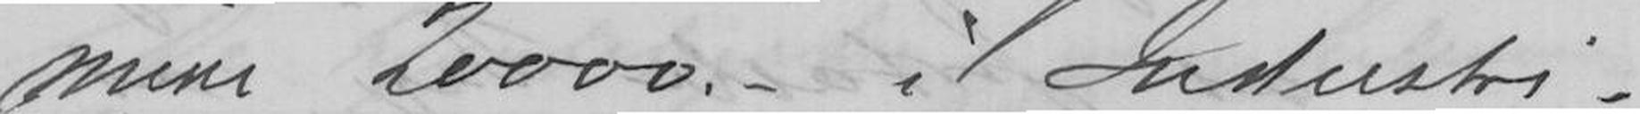mine 20000.- i Industri-
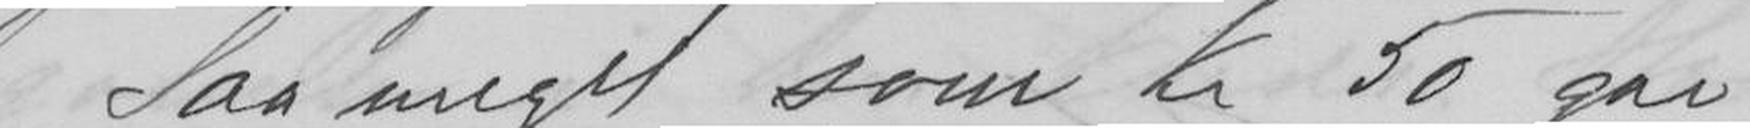Saa meget som kr 50 gav
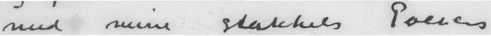med mine stakkels Poesens
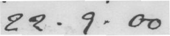22.9.00
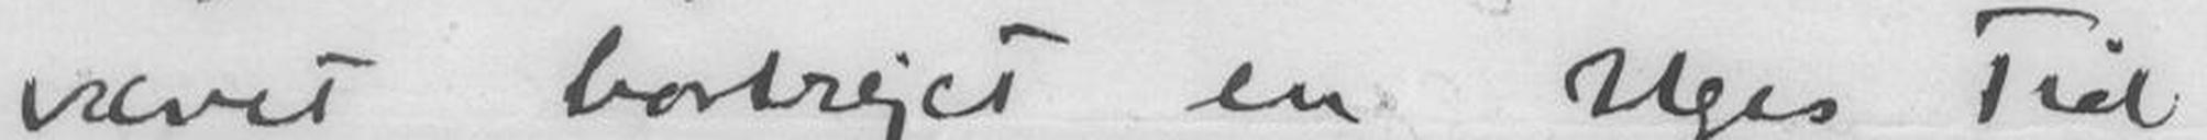været bortrejst en Uges Tid,
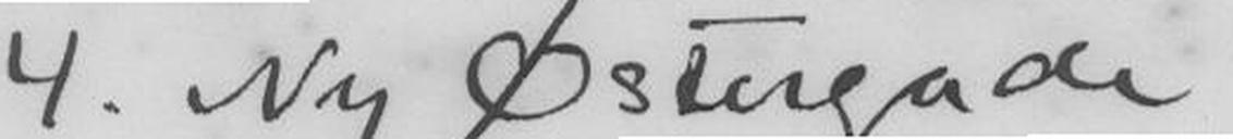4. Ny Østergade
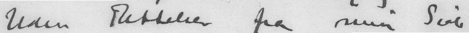Uden Rettelser fra min Side,
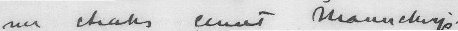nu straks sendt Manuchirp-
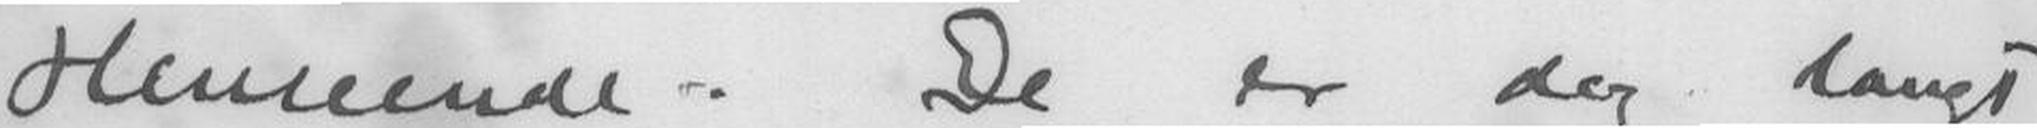Henseende. De er dog langt
